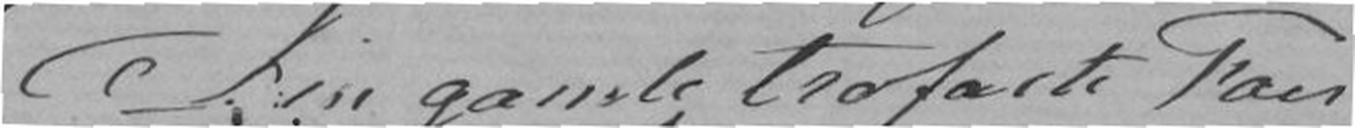Din gamle trofaste Faer
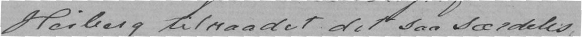Heiberg tilraadet det saa særdeles
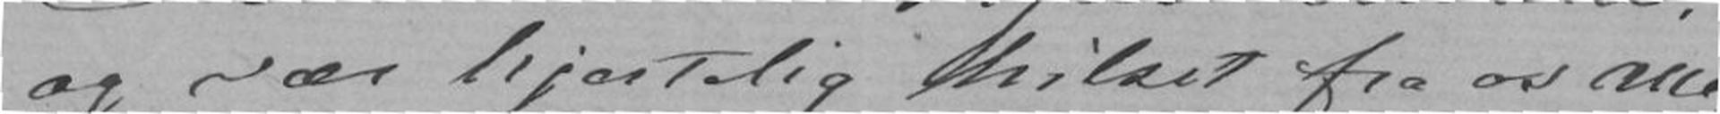og vær hjertelig hilset fra os Alle.
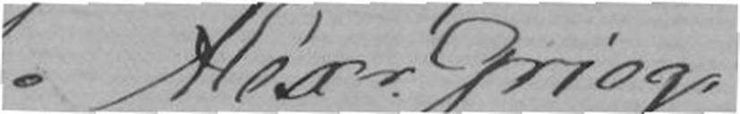Alexr. Grieg.
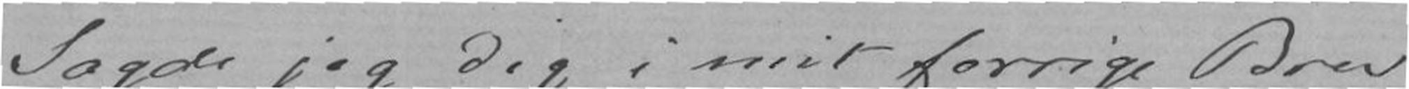Sagde jeg Dig i mit forrige Brev
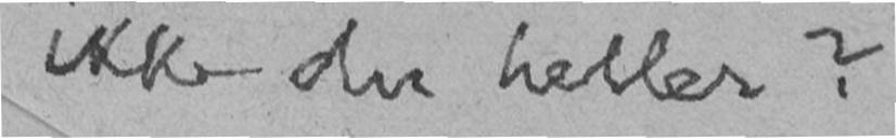ikke du heller?
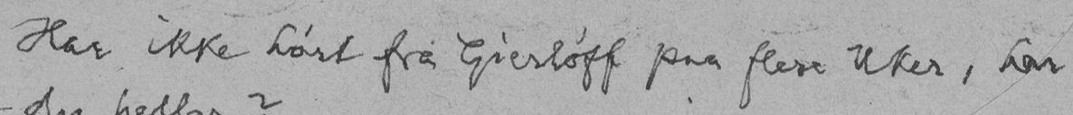Har ikke hørt fra Gierløff paa flere Uker, har
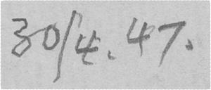30/4. 47.
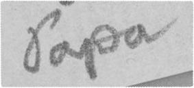Papa
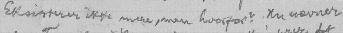Eksisterer ikke mere, men hvorfor? Nu nævner
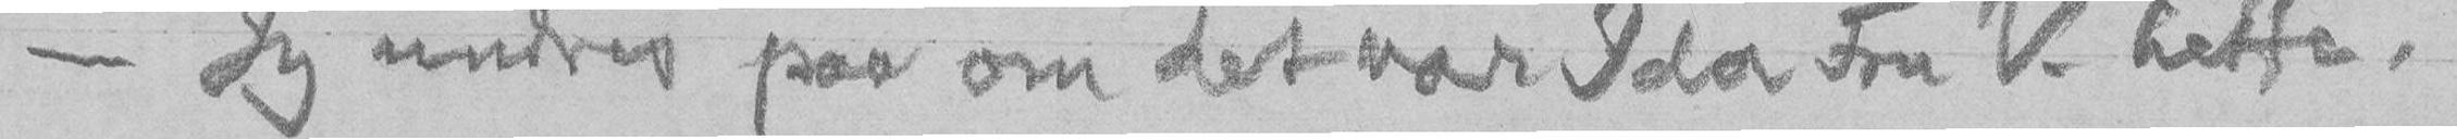- Jeg undres paa om det var Ida Fru V. hette.
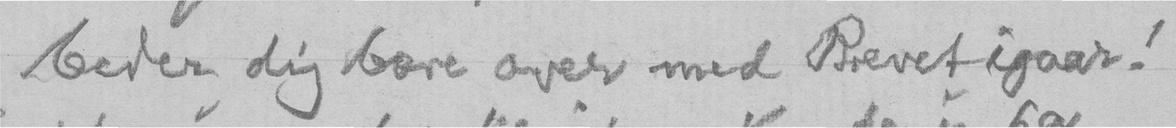beder dig bære over med Brevet igaar!
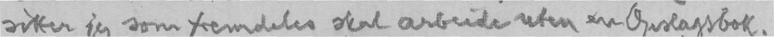sitter jeg som fremdeles skal arbeide uten en Opslagsbok.
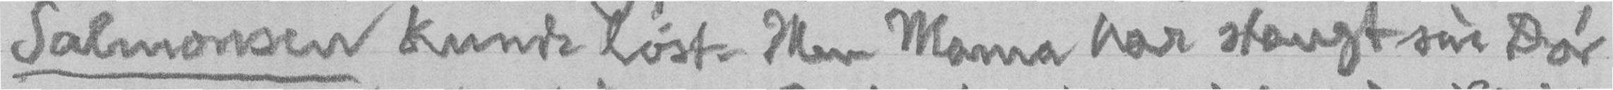Salmonsen kunde løst. Men Mama har stængt sin Dør
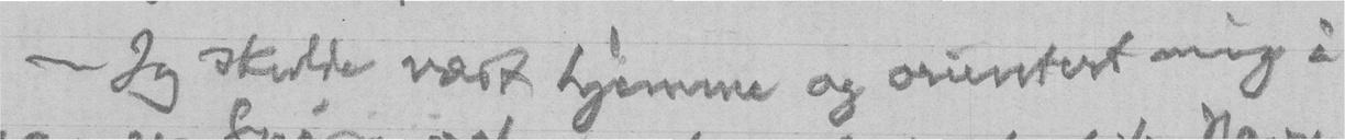- Jeg skulde vært hjemme og orientert mig i
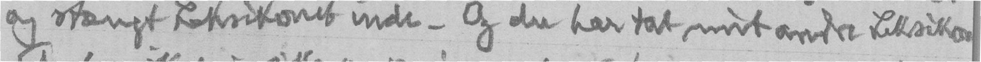og stængt Leksikonet inde. Og du har tat mit andre Leksikon
